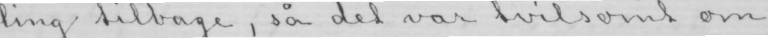ling tilbage, så det var tvilsomt om
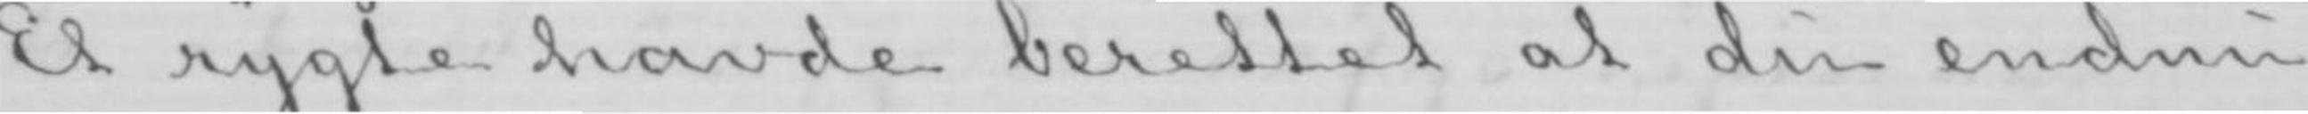Et rygte havde berettet at du endnu
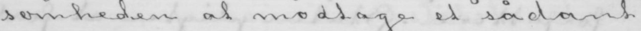somheden at modtage et sådant
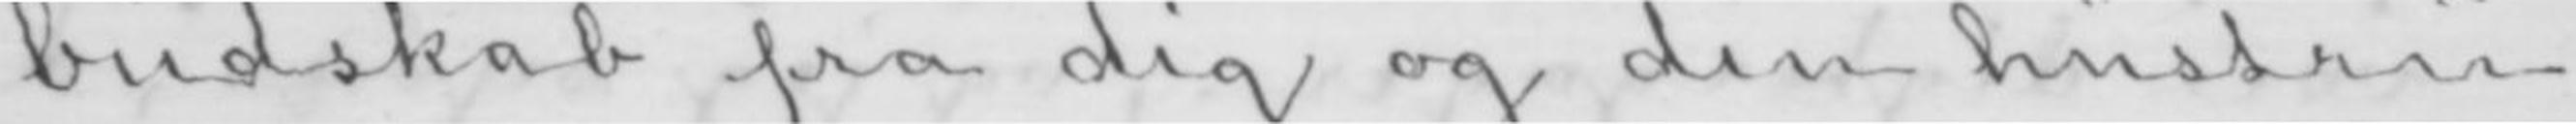budskab fra dig og din hustru
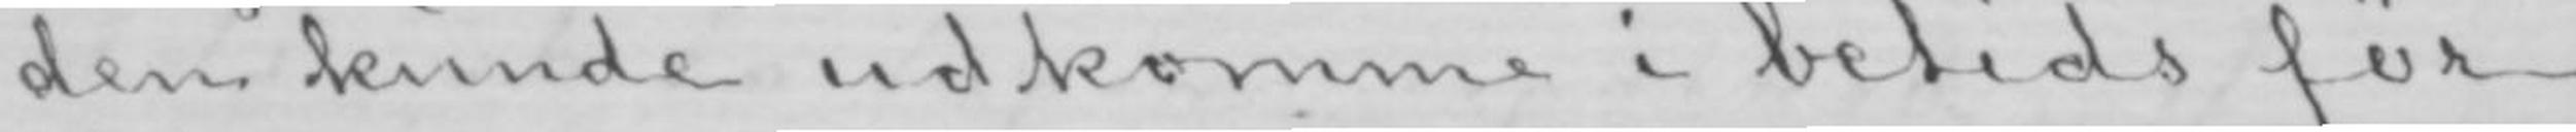den kunde udkomme i betids før
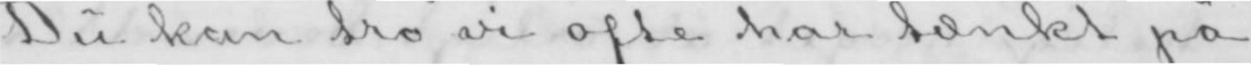Du kan tro vi ofte har tænkt på
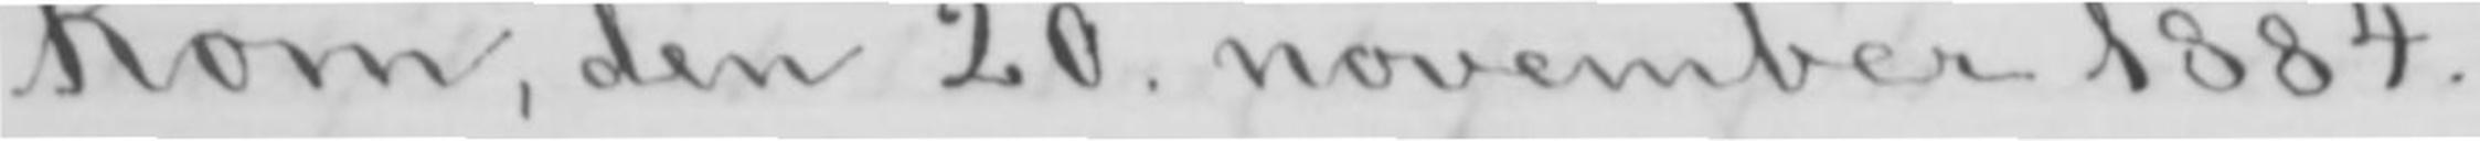Rom, den 20. november 1884.
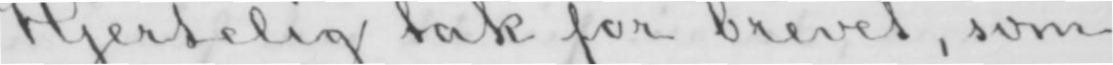Hjertelig tak for brevet, som
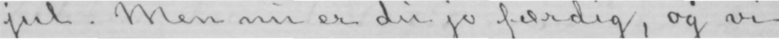jul. Men nu er du jo færdig, og vi
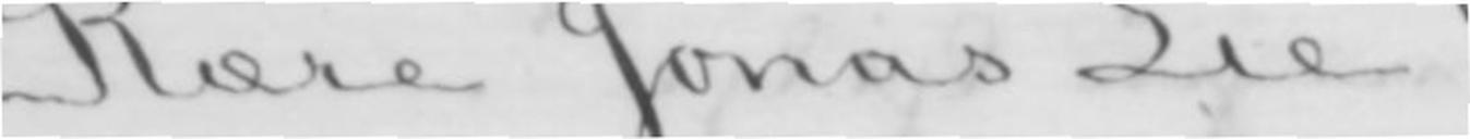Kære Jonas Lie!
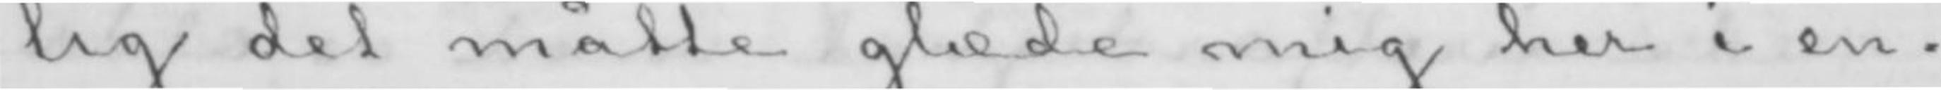lig det måtte glæde mig her i en-
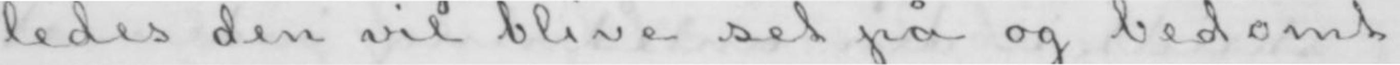ledes den vil blive set på og bedømt
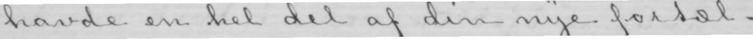havde en hel del af din nye fortæl-
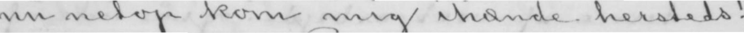nu netop kom mig ihænde hersteds!
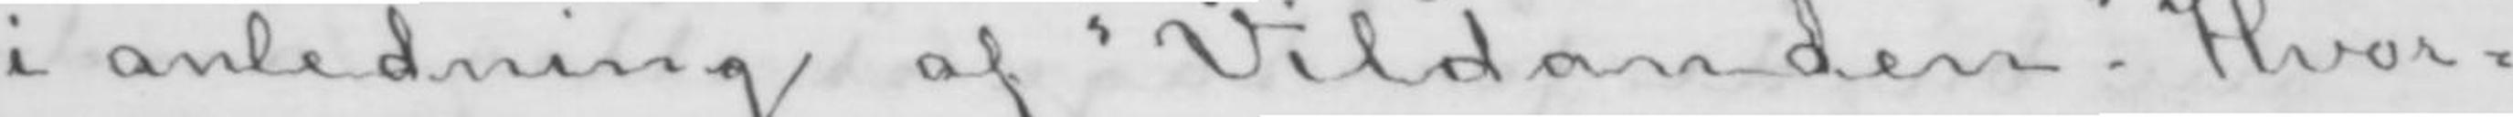i anledning af «Vildanden». Hvor-
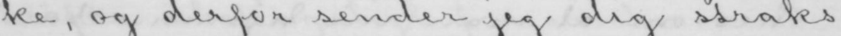ke, og derfor sender jeg dig straks
